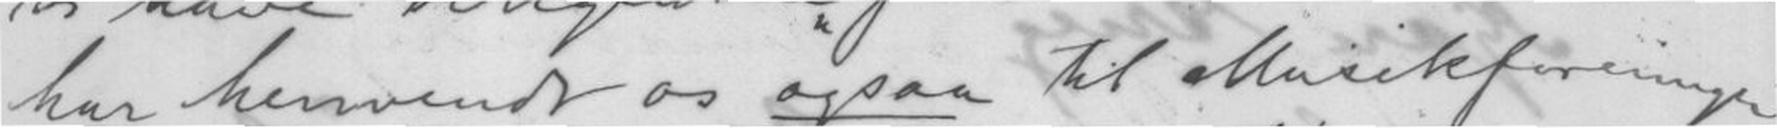har henvendt os ogsaa til Musikforeningen
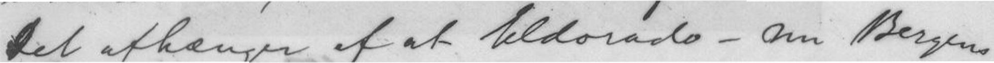Det afhænger af at Eldorado - nu Bergens
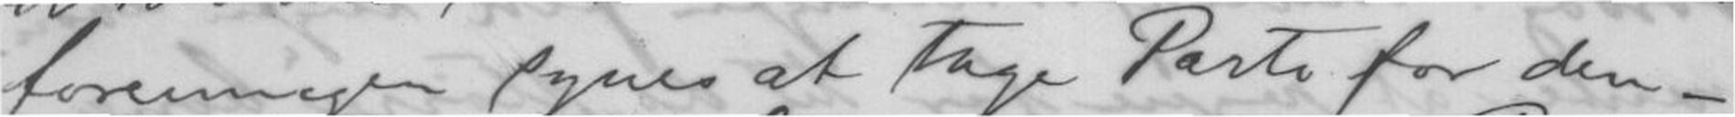foreningen synes at tage Parti for den -
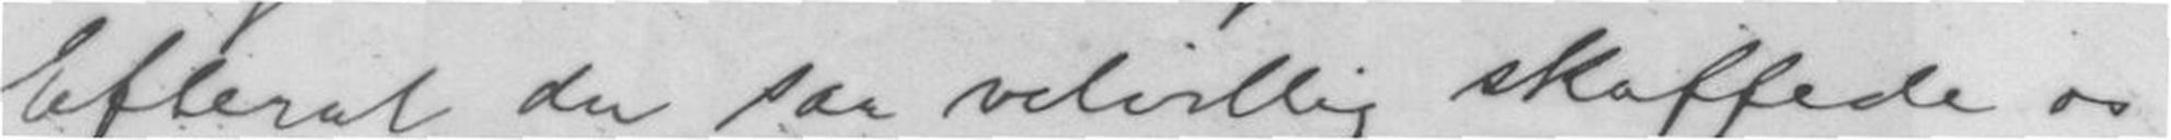Efterat du saa velvillig skaffede os
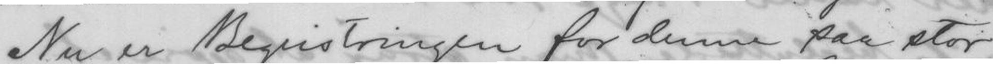Nu er Begeistringen for denne saa stor
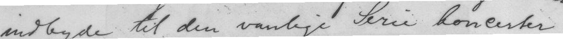indbyde til den vanlige Serie Concerter
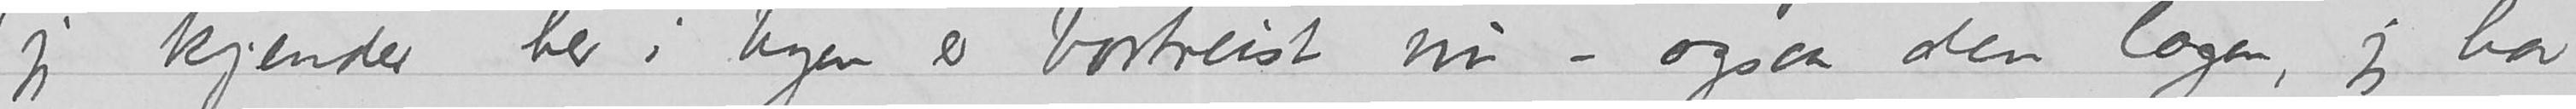jeg kjender her i byen er bortreist nu – ogsaa den lægen, jeg har
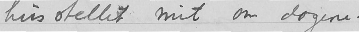husstellet mit om dagene.
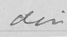din
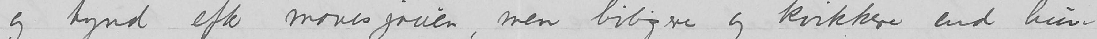og tynd efter <mavesjauen>, men livligere og kvikkere end hun
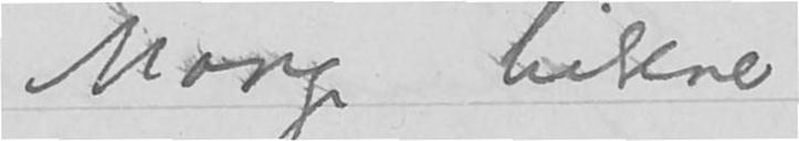Mange hilsener
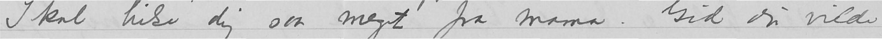Skal hilse dig saa meget fra mama. Giud du vilde
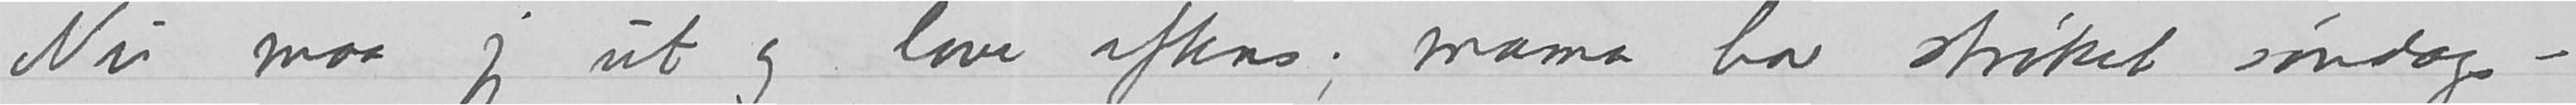Nu maa jeg ut og lave aftens; mama har strøket søndag-
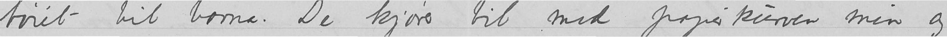støiet til barna. De kjører til med papirkurven min og
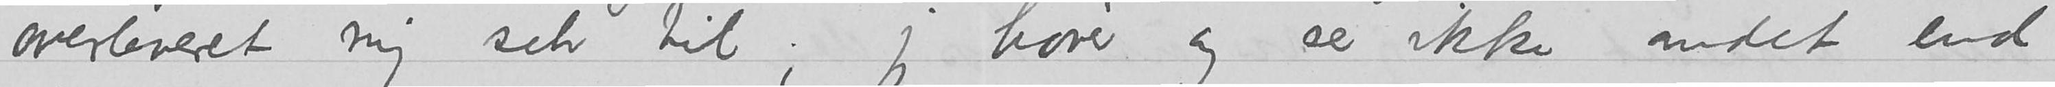overleveret mig selv til; jeg hører og ser ikke andet end
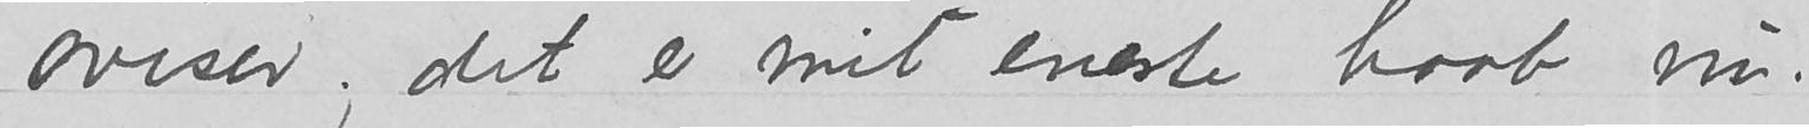aviser; det er mit eneste haab nu.
